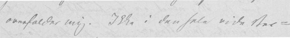overfalder mig. Ikke i den hele vide Ver-
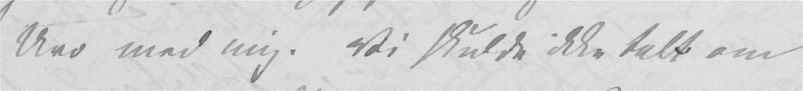Uro med mig. Vi skulde ikke talt om
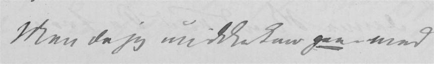Men da jeg nu ikke kan gaa med
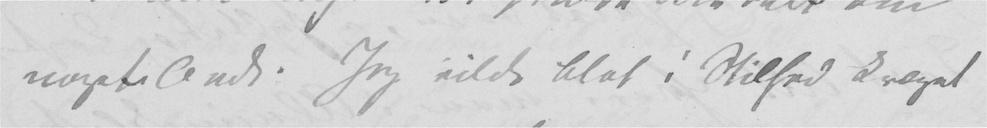noget Ondt. Jeg vilde blot i Stilhed kvæget
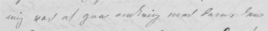mig ved at gaa omkring med Dem, den
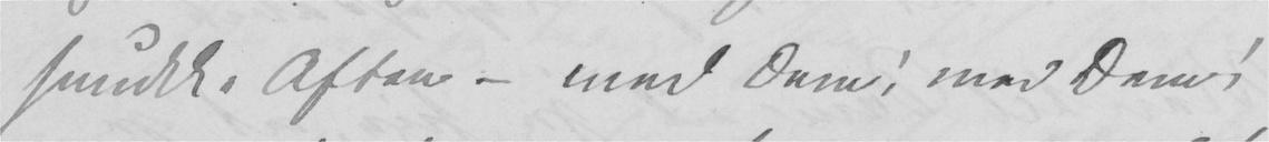smukke Aften – med Dem! med Dem!
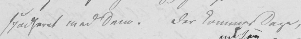spadseret med Dem. Der kommer Dage,
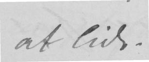at lide.
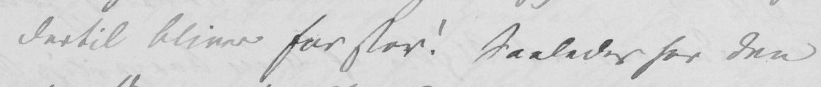dertil bliver for stor! Saaledes har den
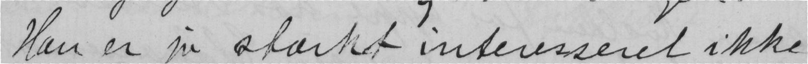Han er jo stærkt interesseret ikke
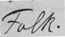Folk.
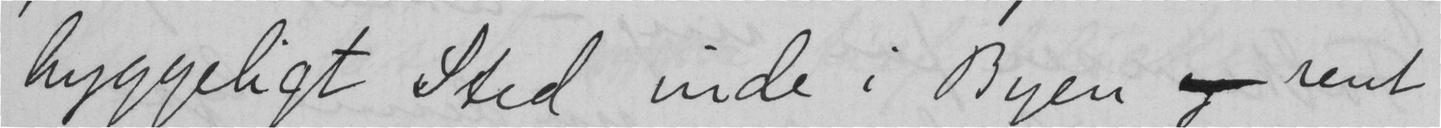hyggeligt Sted inde i Byen og rent
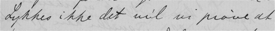Lykkes ikke det vil vi prøve at
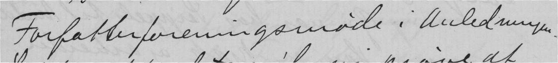Forfatterforeningsmøde i Anledningen.
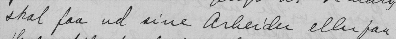skal faa ud sine arbeider eller faa
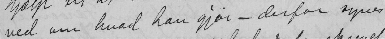ved om hvad han gjør - derfor synes
